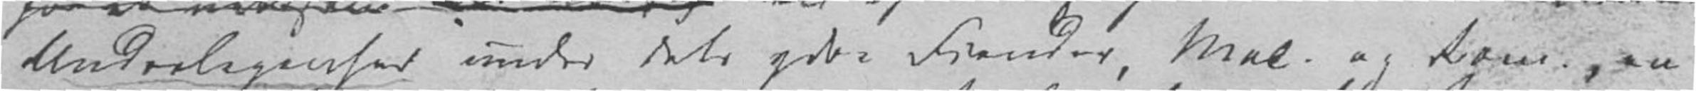Underlegenhed under dets ydre Fiender Mel. og Rom., en
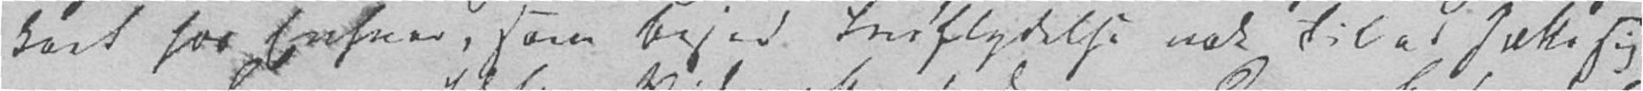kort hos Enhver, som besad Indflydelse nok til at sætte sig
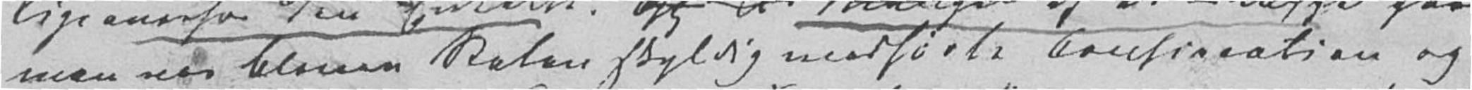man var bleven Staten skyldig medførte Confiscation og
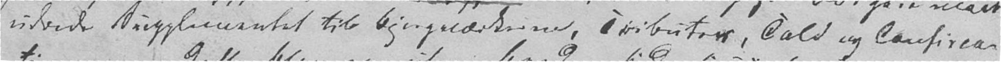udrede Supplementet til Bjergværkerne, Tributes, Told og Confisca-
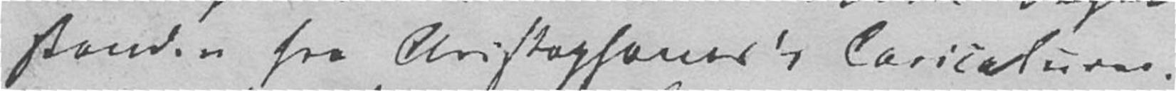standen fra Aristophanes's Caricaturer.
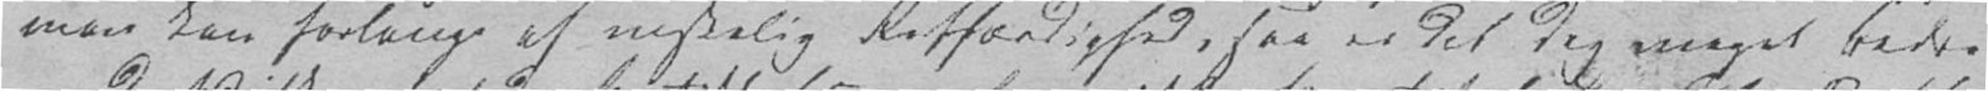man kan forlange af mnskelig Retfærdighed, saa er det dog meget bedre
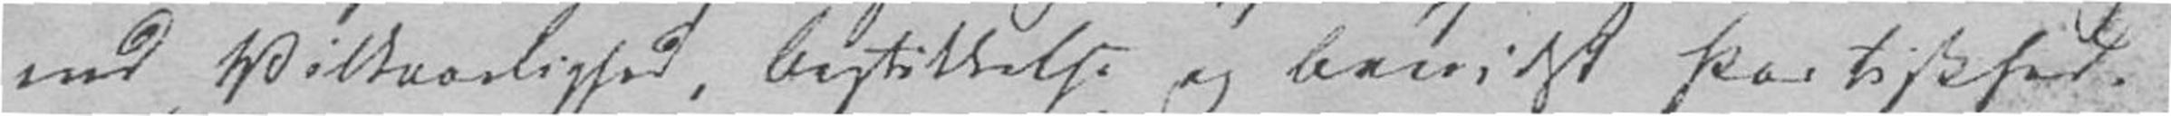end Vilkaarlighed, Bestikkelse og bevidst Partiskhed.
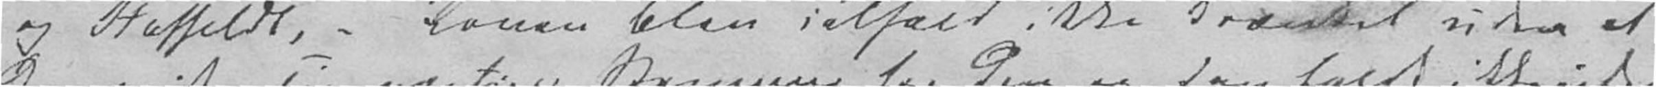og Staffeldt, – Loven blev ialfald ikke krænket uden at
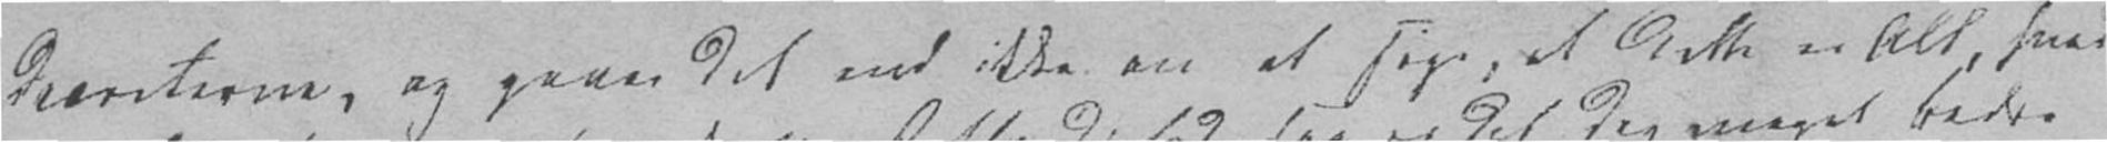decreterne, og gaaer det end ikke an at sige, at dette er Alt, hvad
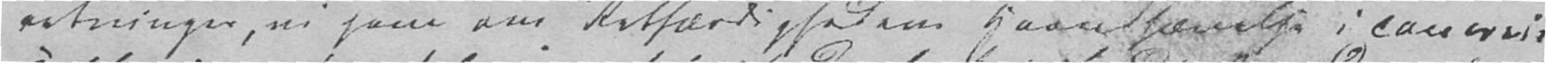retninger, vi have om Retfærdighedens Haandhævelse i concrete
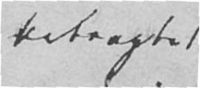betragtet
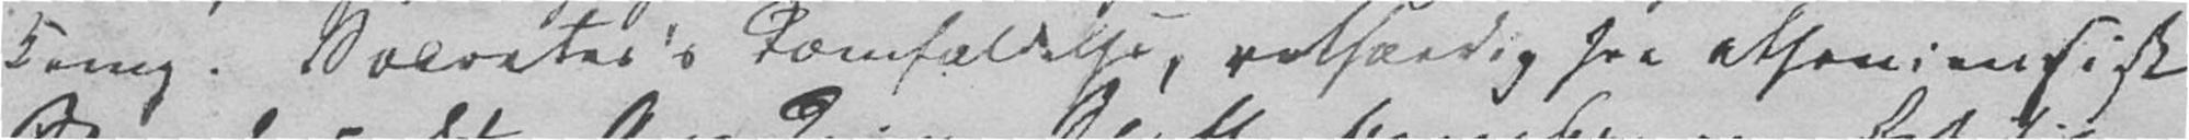Kamp. Socrates's Domfældelse, retfærdig fra atheniensisk
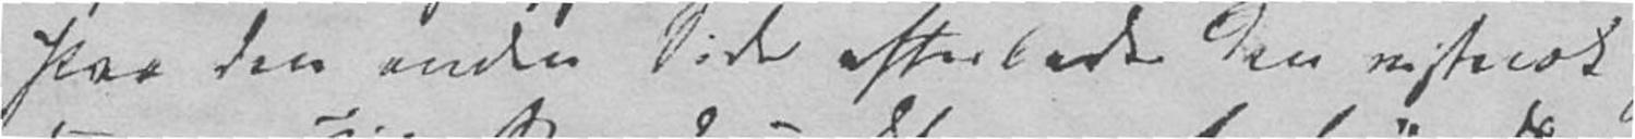Paa den anden Side efterlader den vistnok
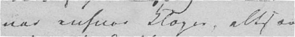var enhver Klager, altsaa
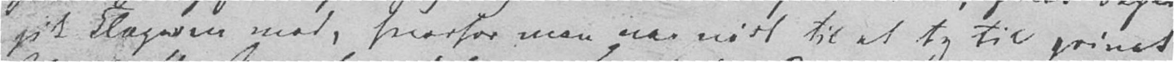gik Klageren mod, hvorfor man var nødt til at ty til privat
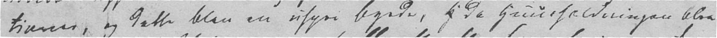tioner, og dette blev en uhyre Byrde, da Huusholdningen blev
